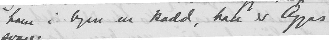ham i byen en kveld, han er Agges
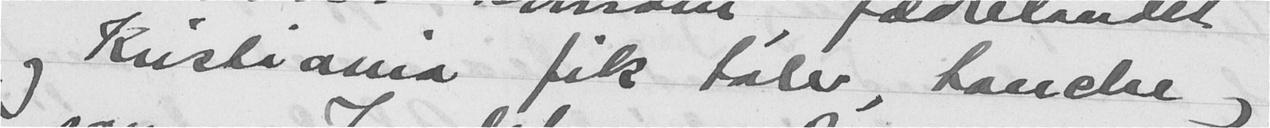og Kristiania fik taler, tanelse og
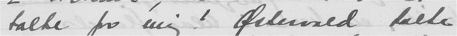talte for mig! Østevold talte
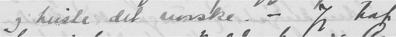og heiste det norske. - Jeg traf
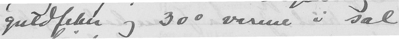guldfeber og 30º varme i sal
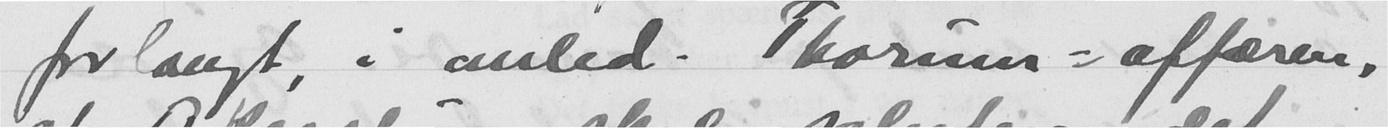forlangt, i anled. Thorum-affæren
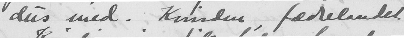dus med. Kvinden, fædrelandet
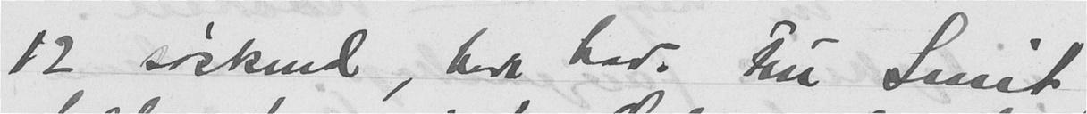12 søskend, han har. Fru Smit
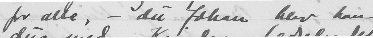for alle, - du Johan blev han
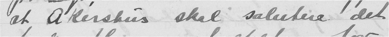at Akershus skal salutere det
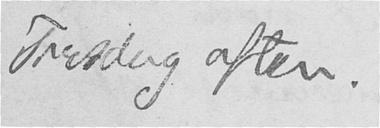Tirsdag aften.
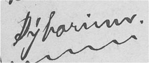þýborinn.
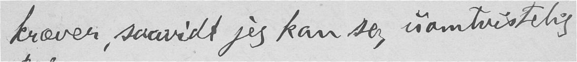kræver, saavidt jeg kan se, uomtvistelig
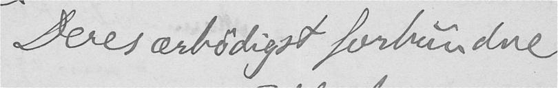Deres ærbødigst forbundne
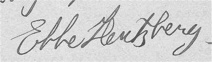Ebbe Hertzberg.
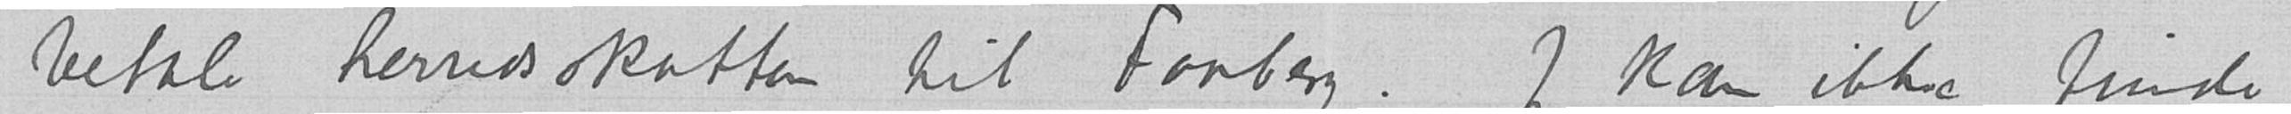betale herredsskatten til Faaberg. Jeg kan ikke finde
